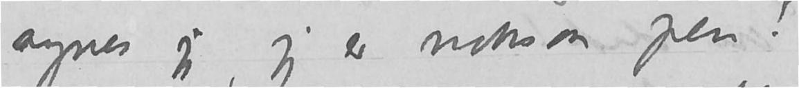synes jeg, jeg er noksaa pen!
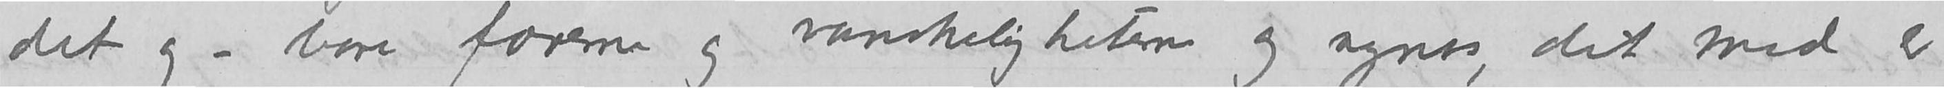det og – bare farerne og vanskeligheterne og synes, det med er
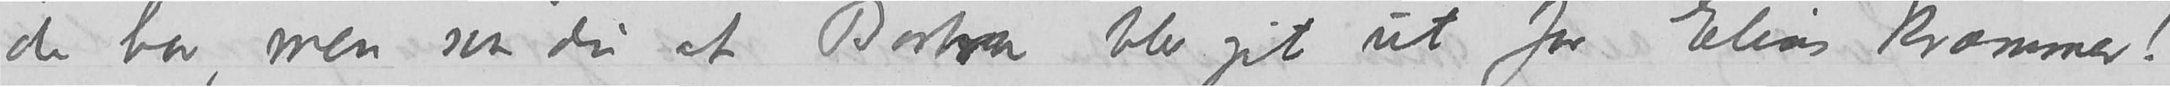de har, men saa du at Barbra blev git ut for Elias Kræmmer!
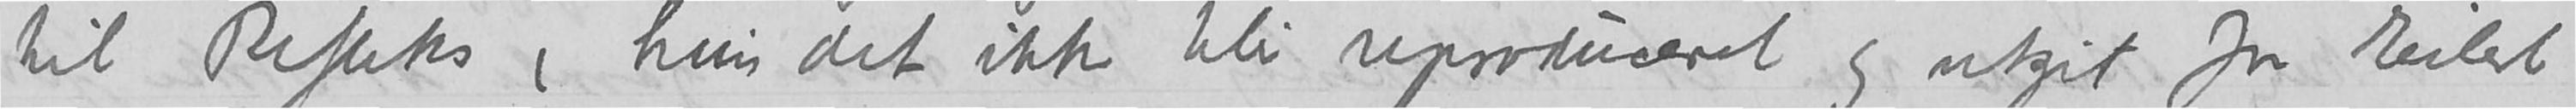til Refleks (hvis det ikke blir reproduceret og utgit for Eilert
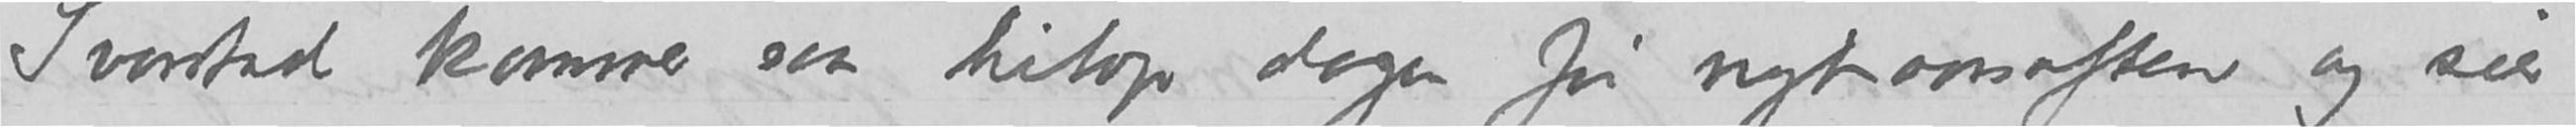Svarstad kommer saa hitop dagen før nytaarsaften og sier
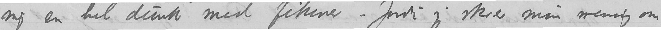mig en hel dunk med fikener – fordi jeg skrev min mening om
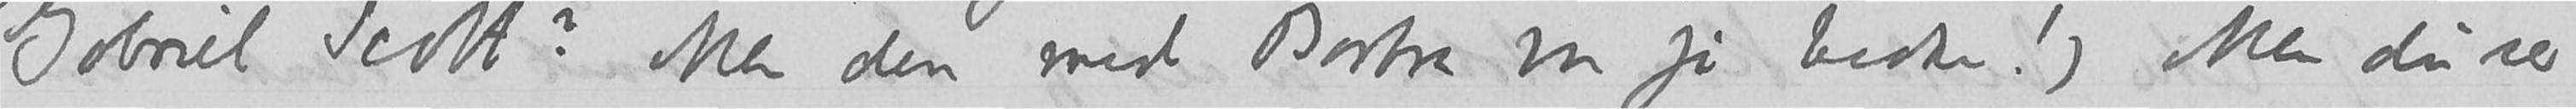Gabriel Scott? Men den med Barbra var jo bedste!) Men du ser
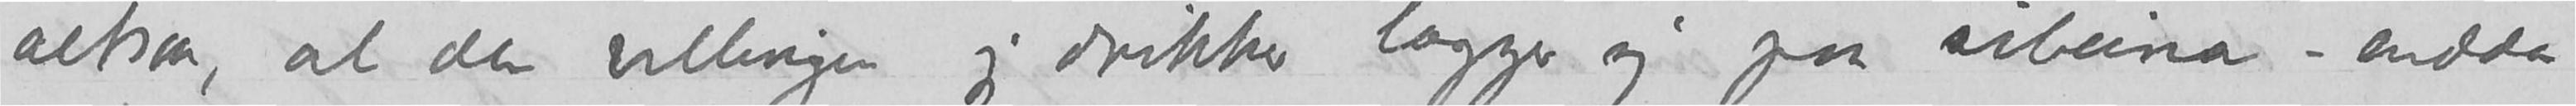altsaa, al den vellingen jeg drikker lægger sig paa ribeina – endda
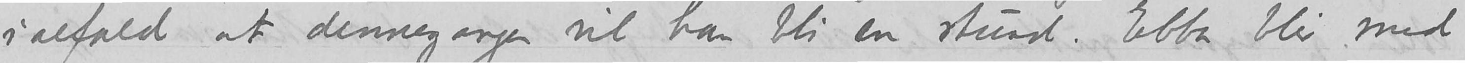ialfald at dennegangen vil han bli en stund. Ebba blir med
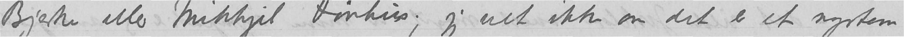Bjerke eller Mikkjel Fønhus; jeg vet ikke om det er et system
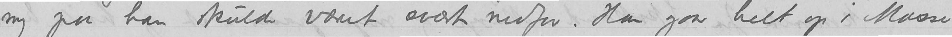mig paa han skulde været svært nedfor. Han gaar helt op i Mosse
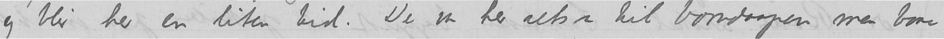og blir her en liten tid. De var her altsaa til barndaapen men bare.
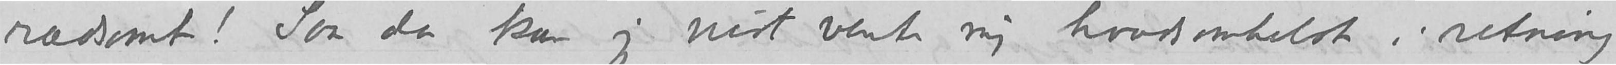rædsomt! Saa da kan jeg vist vente mig hvadsomhelst i retning
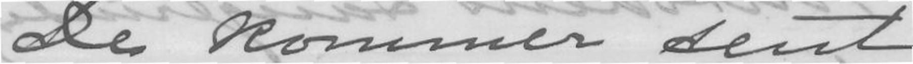De kommer sent;
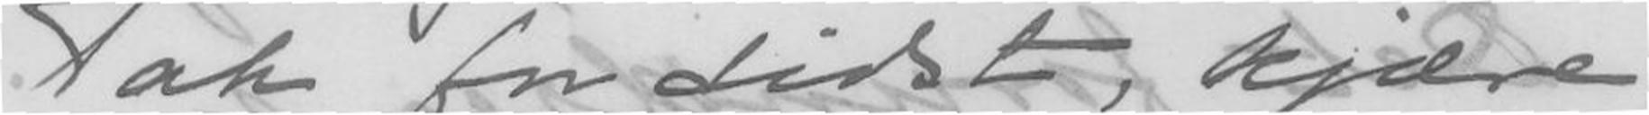Tak for sist, kjære
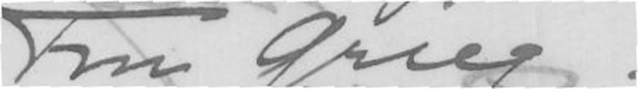Fru Grieg!
